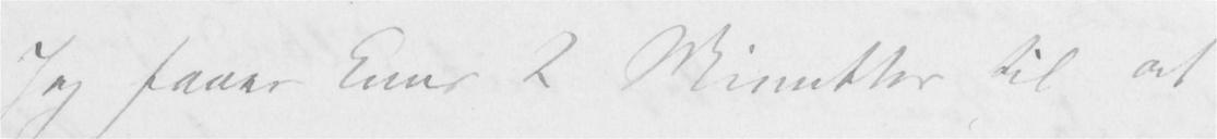Jeg faaer kuns 2 Minutter til at
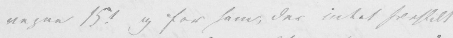regne 15! og for ham, der intet særskilt
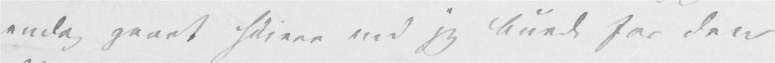endog gaaet høiere end jeg burde for den
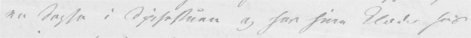en Sopha i Spisestuen og har sine Klæder hos
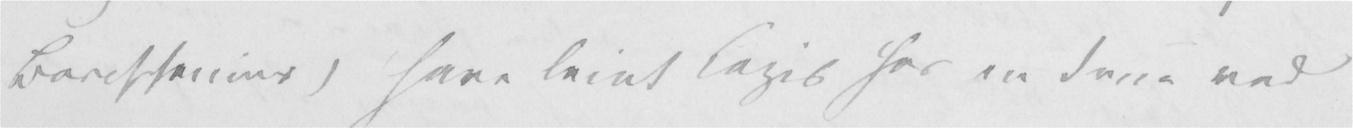Borchsenius) have leiet Logis hos en Frue ved
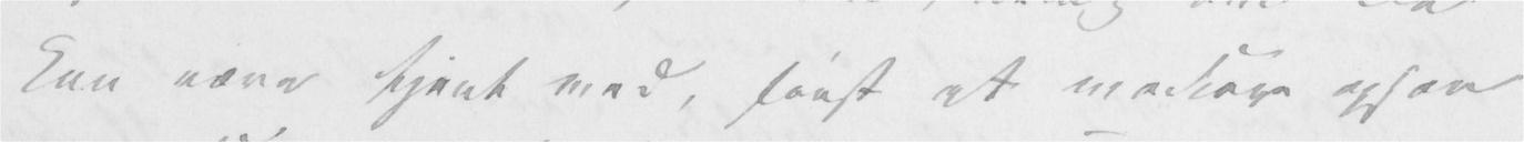kan være tjent med, først at modtage ogsaa
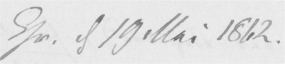Chr. d 19 Mai 1862.
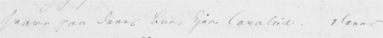svare paa Deres Brev kjere Caroline. Deres
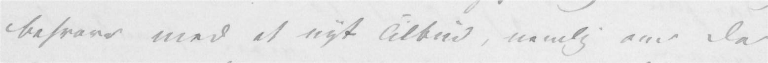besvare med et nyt Tilbud, nemlig om De
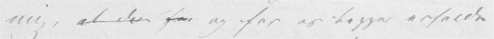mig, at der for og for os begge erholde
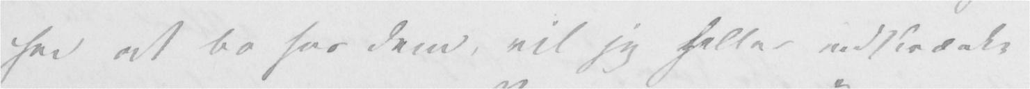hed at bo hos Dem, vil jeg heller indskrænke
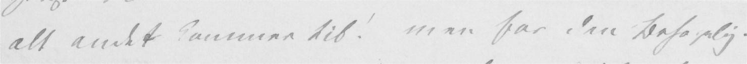alt andet kommer til! men for den Behagelig-
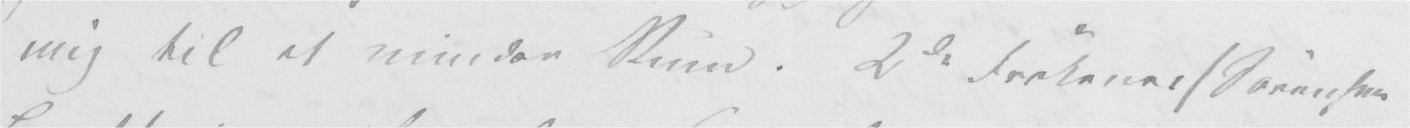mig til et mindre Rum. Tvende Frøkener (Sørensen
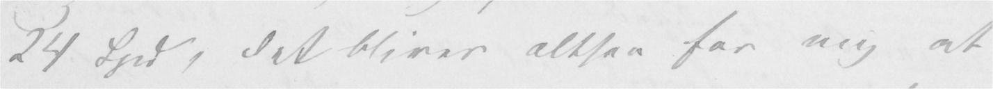24 Spd, det bliver altsaa for mig at
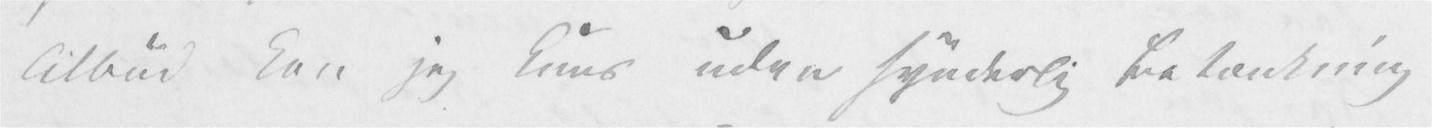Tilbud kan jeg kuns uden synderlig Betænkning
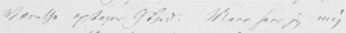Værelse optager, 9 Spd. Mere seer jeg mig
